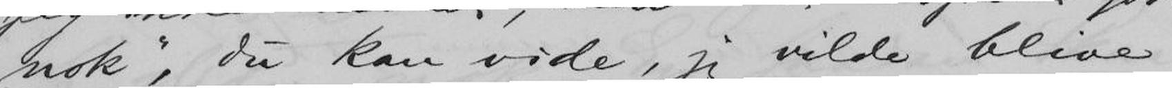nok", du kan vide, jeg vilde blive
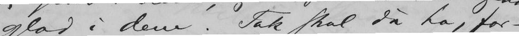glad i dem. Tak skal du ha, for-
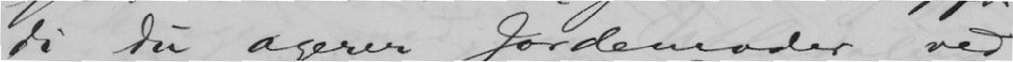di du agerer Jordemoder ved
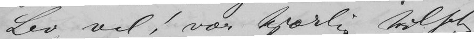Lev vel! vær kjærlig hilset
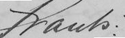Frants
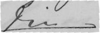Din
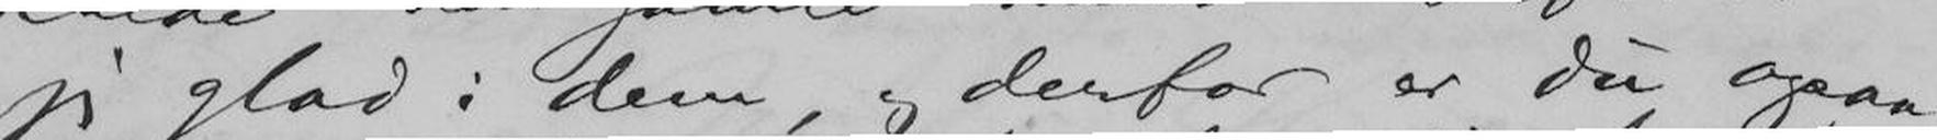jeg glad i dem, og derfor er du ogsaa
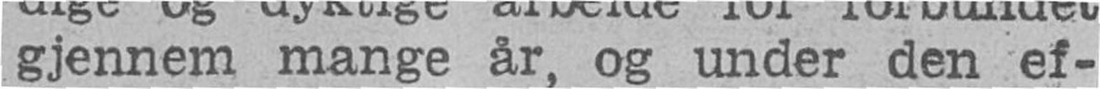gjennem mange àr, og under den ef-
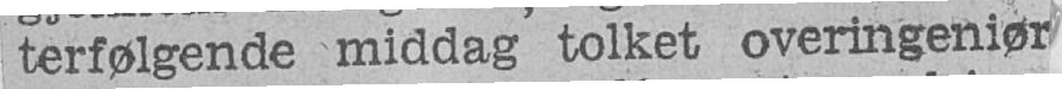terfølgende middag tolket overingeniør
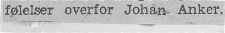følelser overfor Johan Anker.
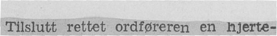Tilslutt rettet ordføreren en hjerte-
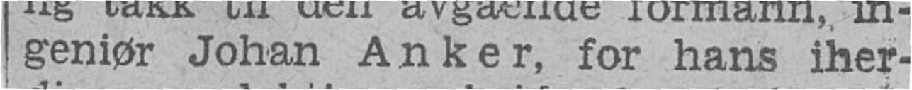geniør Johan Anker, for hans iher-
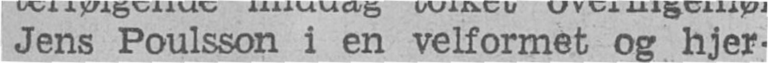Jens Poulsson i en velformet og hjer-
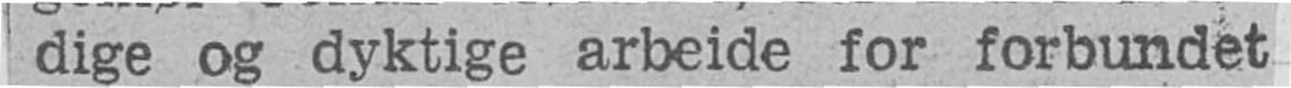dige og dyktige arbeide for forbundet
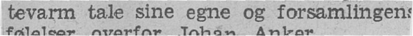tevarm tale sine egne og forsamlingens
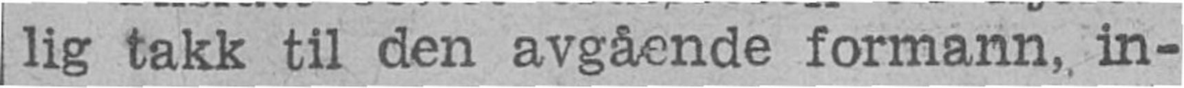lig takk til den avgående formann, in-
